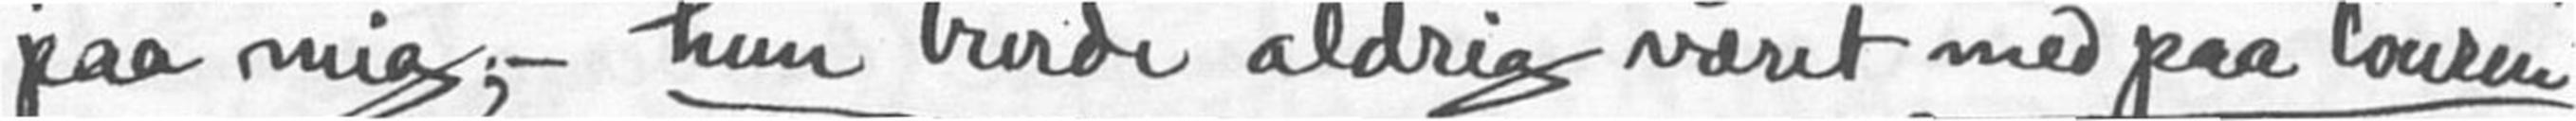paa mig; - hun burde aldrig været med paa touren!
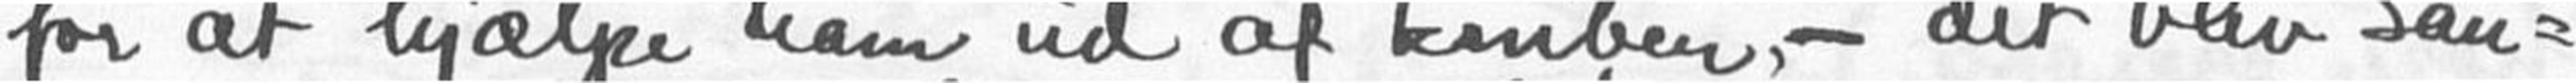for at hjælpe ham ud af kniben,- det blev san-
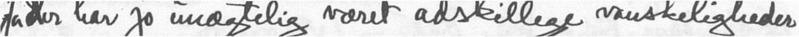Ja der har jo unægtelig været adskillige vanskeligheder
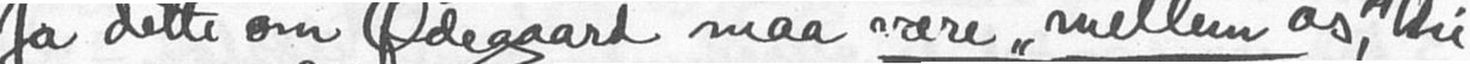Ja dette om Ødegaard maa være "mellem os", thi
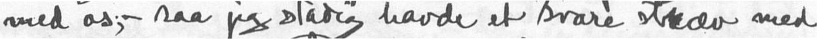med os; - saa jeg stadig havde et svare stræv med
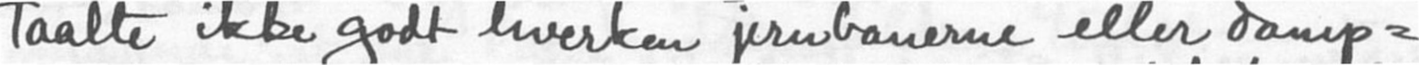taalte ikke godt hverken jernbanerne eller damp-
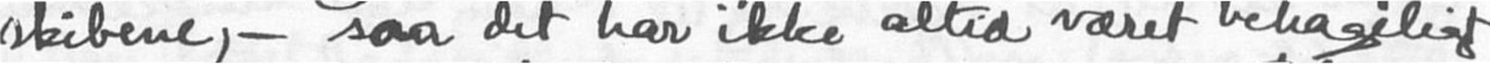skibene, - saa det har ikke altid været behageligt
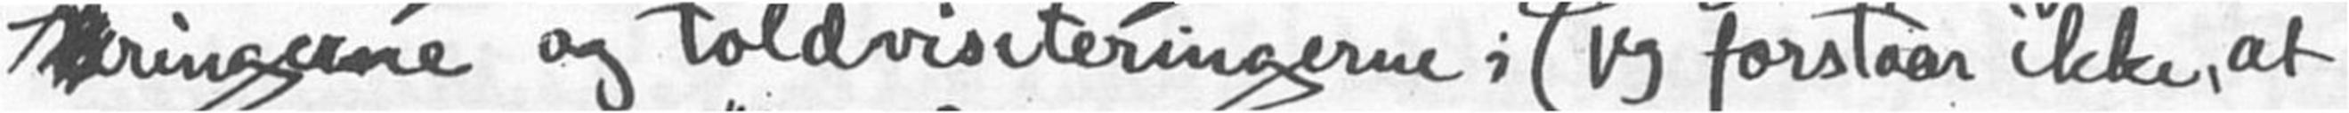ringerne og toldvisiteringrene; (jeg forstaar ikke, at
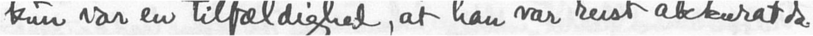kun var en tilfældighed, at han var reist akkurat da.
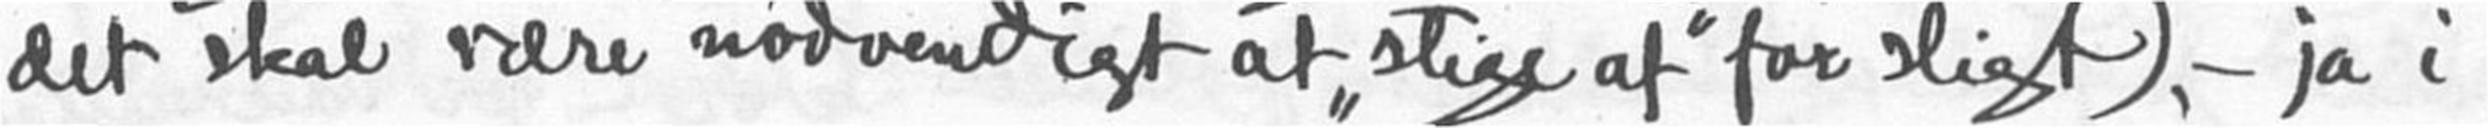det skal være nødvendigt at "stige af" for sligt), - ja i
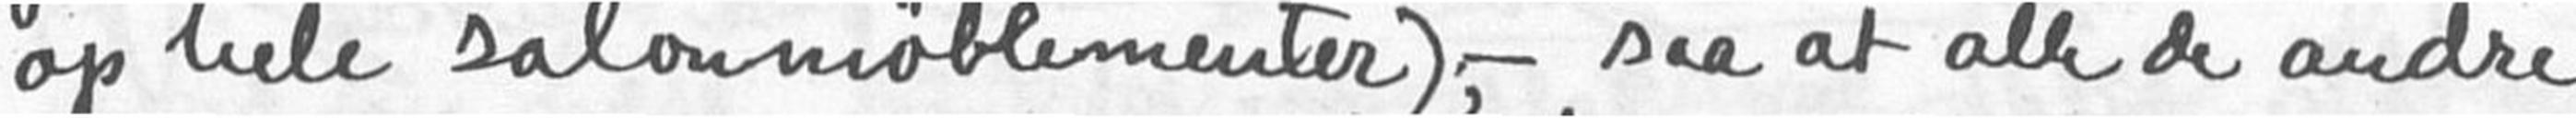op hele salonmøblementer), - saa at alle de andre
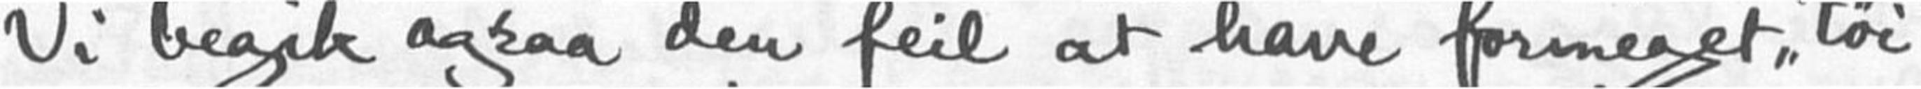Vi begik ogsaa den feil at have formeget "tøi"
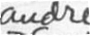andre
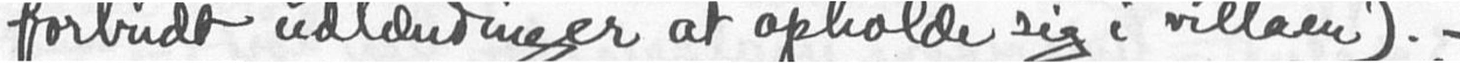forbudt udlændinger at opholde sig i villaen). -
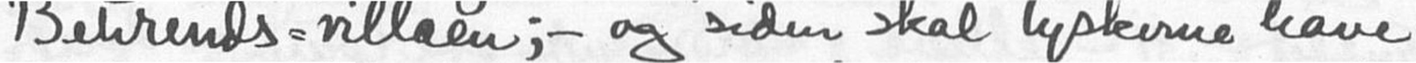Behrends-villaen; - og siden skal tyskerne have
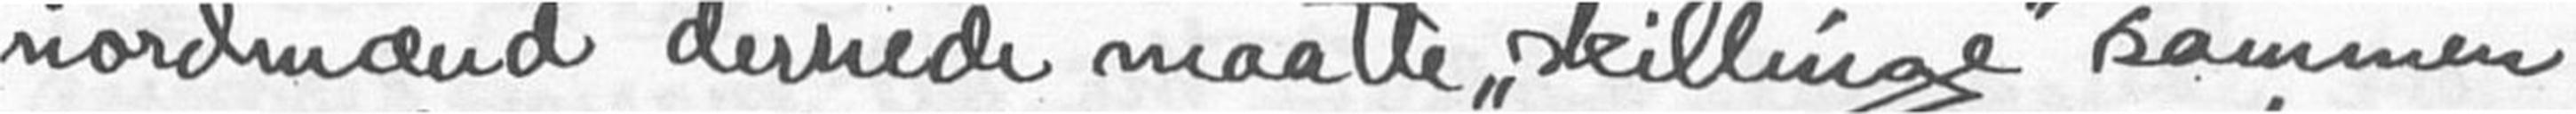nordmænd dernede maatte "skillinge" sammen
